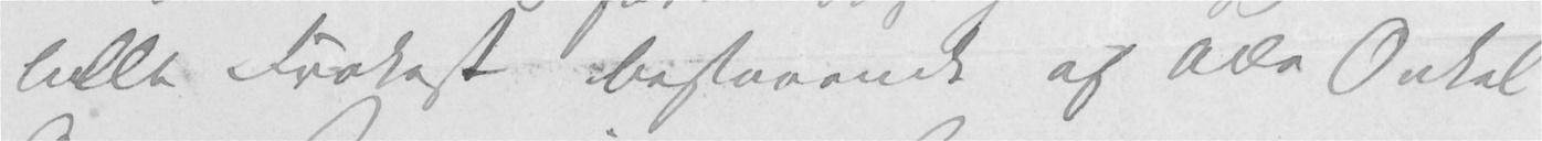lille Frokost bestaaende af alle Onkel
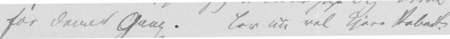for denne Gang. Lev nu vel kjere Robert.
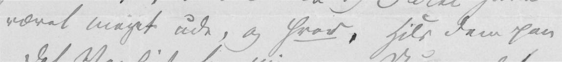været meget ude, og hvor. Hils dem paa
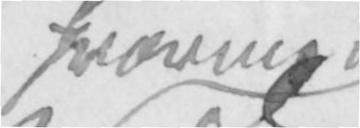hvormed
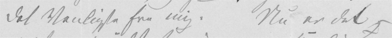det Venligste fra mig. Nu er det
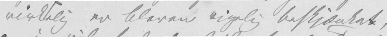virkelig er bleven rigelig beskjænket,
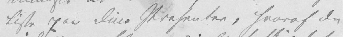Liste paa Dine Presenter, hvoraf Du
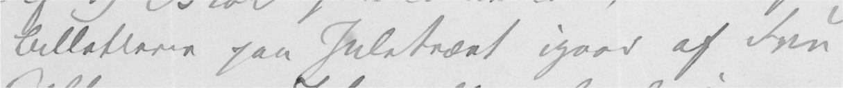Billetterne paa Juletræet igaar af Fru
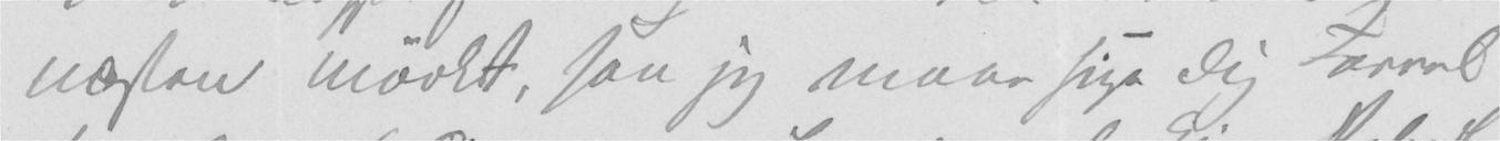næsten mørkt, saa jeg maae sige Dig Farvel
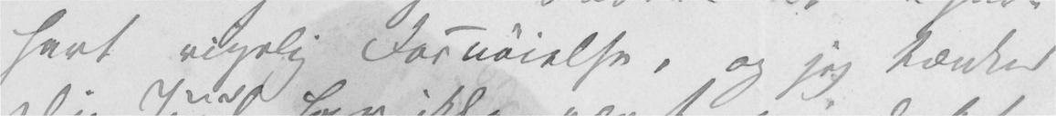havt rigelig Fornøielse, og jeg tænker
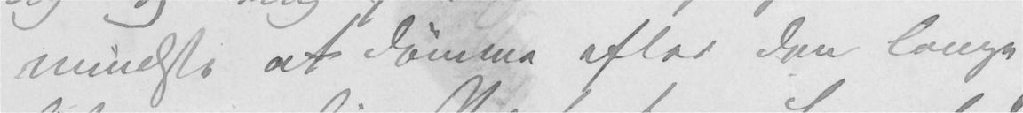mindste at dømme efter den lange
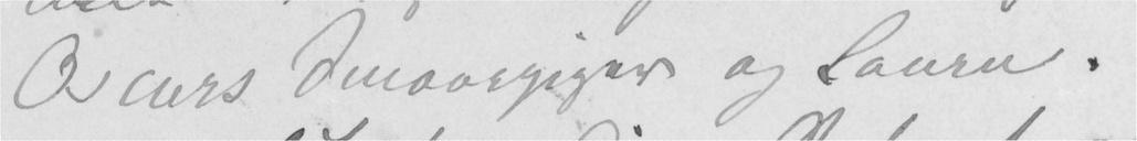Oscars Smaaepiger og Laura.
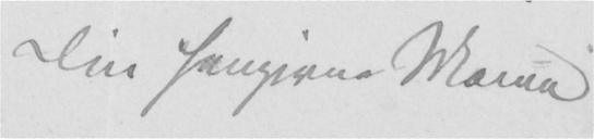Din hengivne Mama
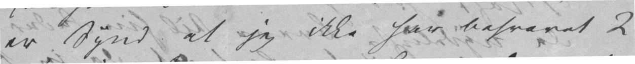er Synd at jeg ikke har besvaret 2
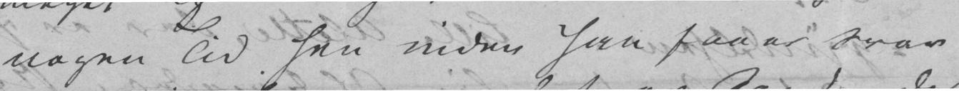nogen Tid hen inden han faaer Svar
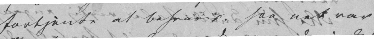fortjente at besvares. saa net var
